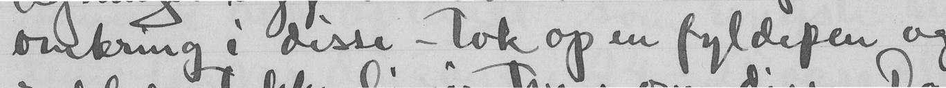omkring i disse - tok op en fyldepen og
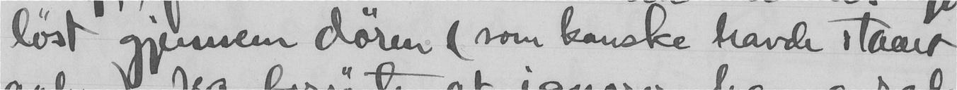løst gjennem døren (som kanske havde staaet
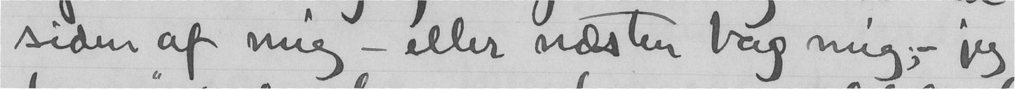siden af mig - eller næsten bag mig; - jeg
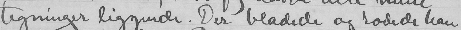tigninger liggende. Der bladede og rodede han
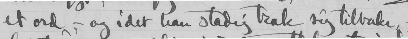et ord, - og idet han stadig trak sig tilbake, -
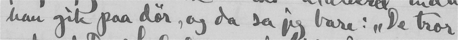han gik paa dør, og da sa jeg bare: "De tror
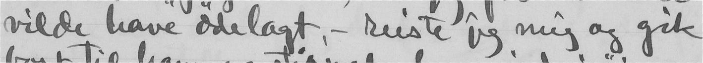vilde have ødelagt, - reiste jeg mig og gik
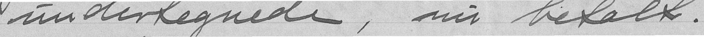undertegnede, nu betalt.
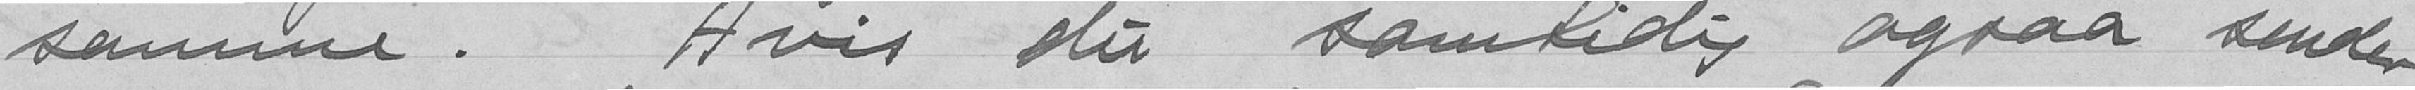samme. Hvis du samtidig ogsaa sender
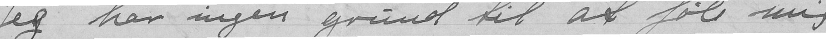Jeg har ingen grundt til at føle mig
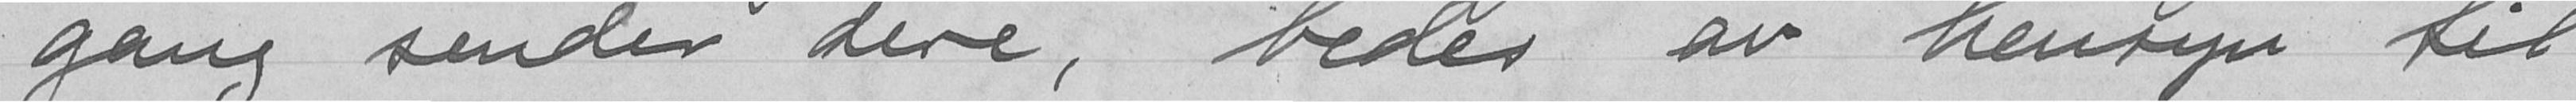gang sender dere, bedes av hensyn til
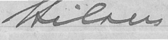Hilsen
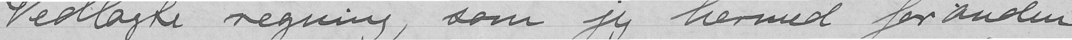Vedlagte regning, som jeg hermed for anden
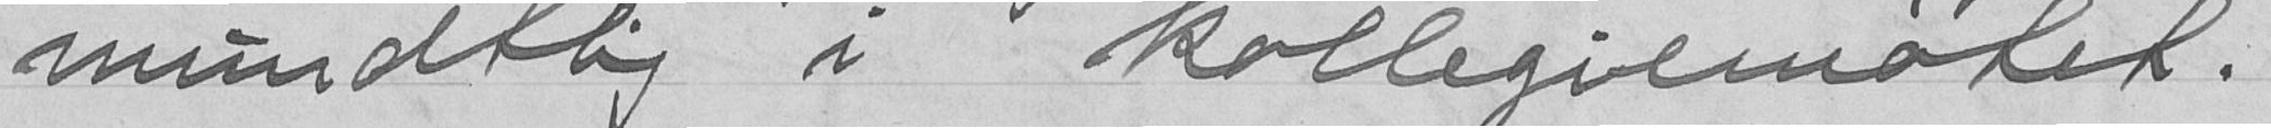mundtlig i kollegiemøtet.
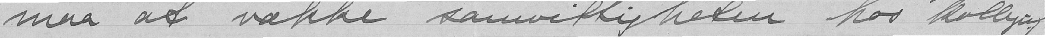maa at vække samvittigheten hos kollegiet
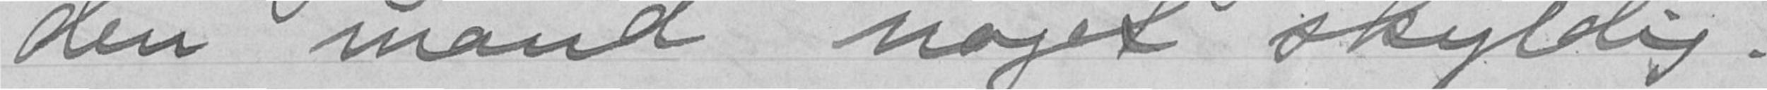den mand noget skyldig.
